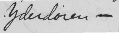Yderdøren -
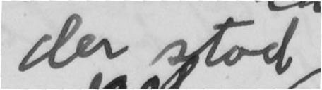der stod
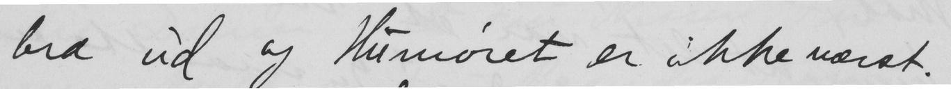bra ud og Humøret er ikke værst.
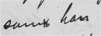som han
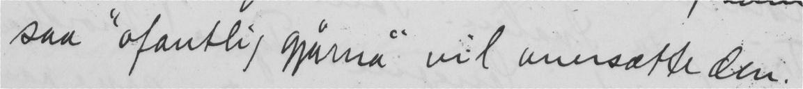saa "ofantlig gjårna" vil oversætte den.
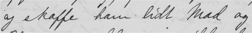og skaffe ham lidt Mad og
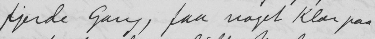fjerde Gang, faa noget Klær paa
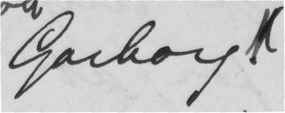Garborg!
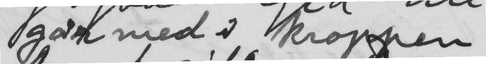går med i kroppen
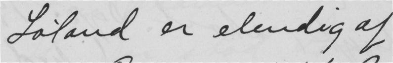Løland er elendig af
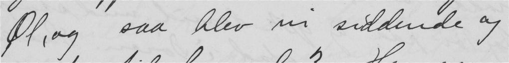Øl, og saa blev vi siddende og
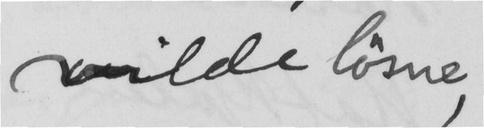vilde løsne,
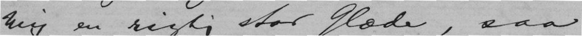mig en rigtig stor Glæde, saa
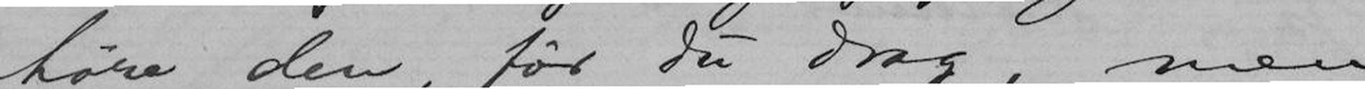høre den, før du drog, men
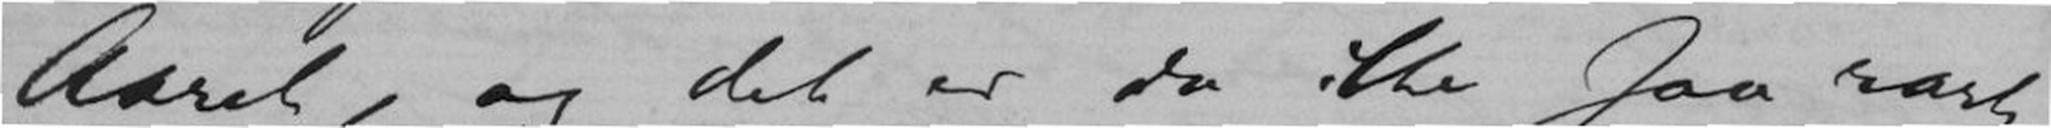Aaret, og det er da ikke saa rart,
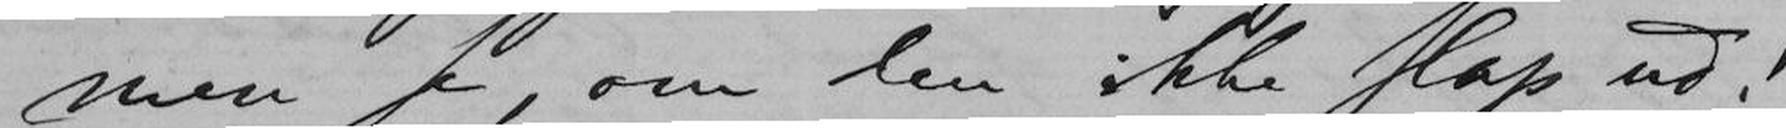men se, om den ikke slap ud!
